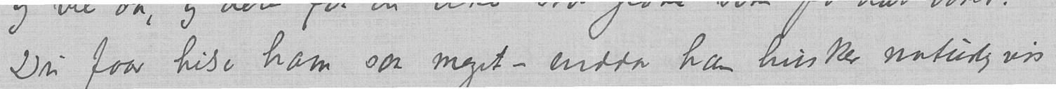Du faar hilse ham saa meget – endda han husker naturligvis
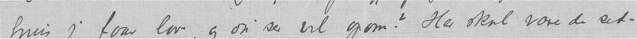hvis jeg faar lov, og du ser vel opom. Her skal være de sed-
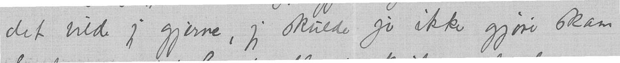det vilde jeg gjerne, jeg skulde jo ikke gjøre skam
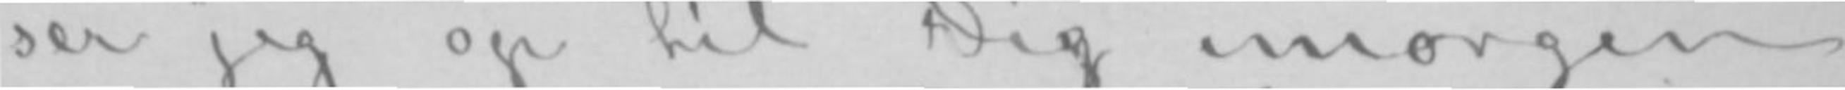ser jeg op til Dig imorgen
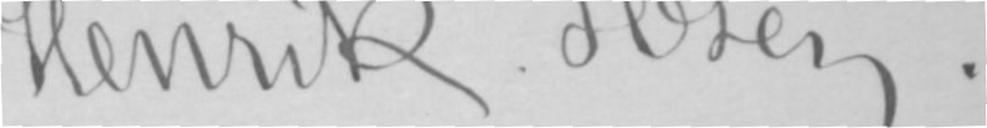Henrik Ibsen.
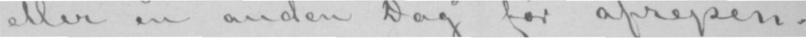eller en anden Dag før afrejsen.
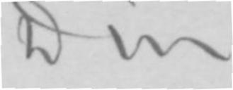Din
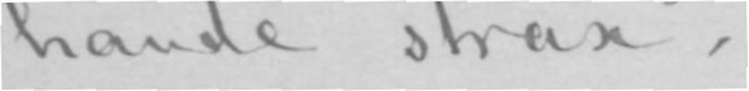hande strax.
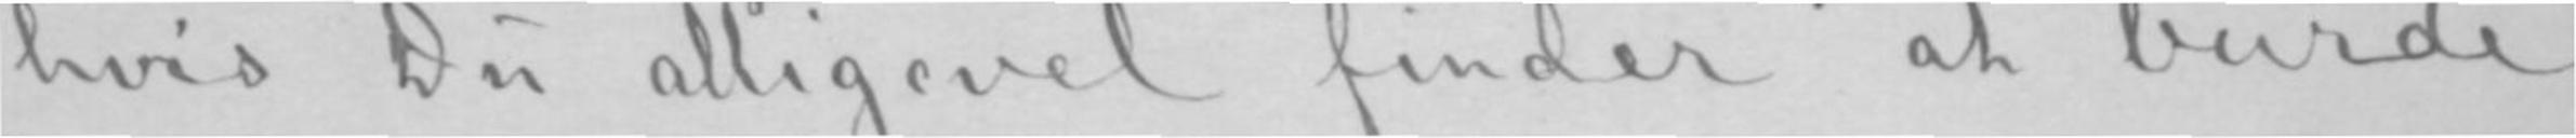hvis Du alligevel finder at burde
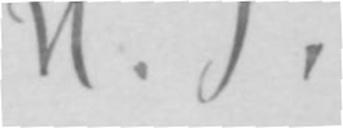H. I.
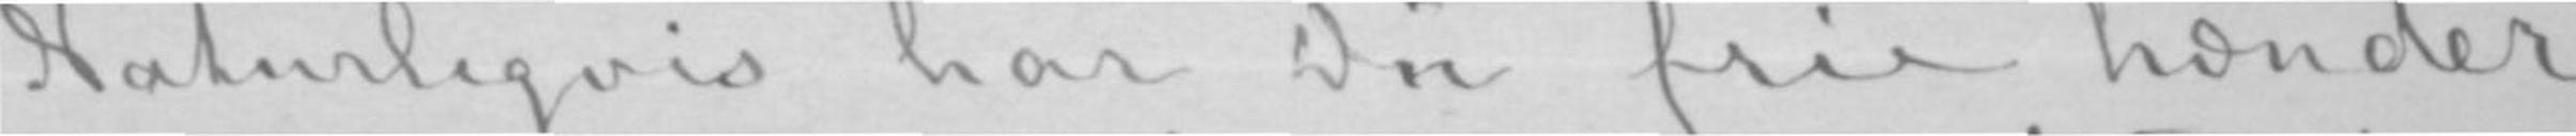Naturligvis har Du frie hænder
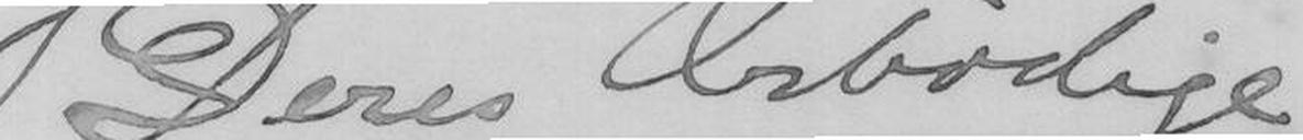Deres Ærbødige
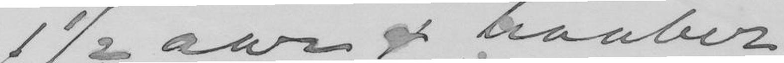1½ aar & haaber
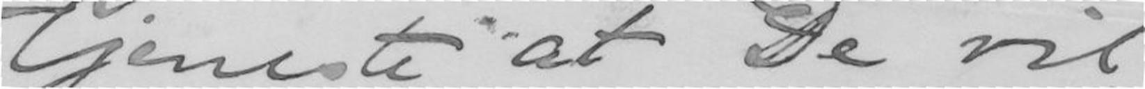tjeneste at De vil
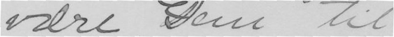være Dem til
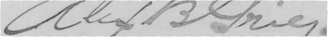Alex B Grieg.
